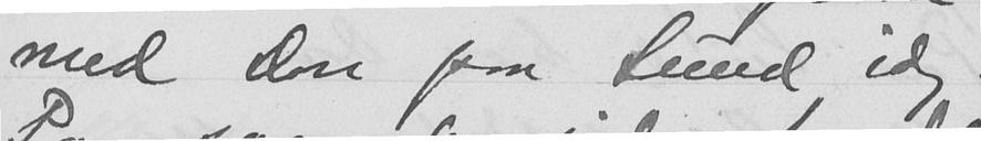med Don paa Sund idag.
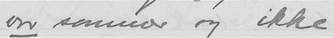var sommer og ikke
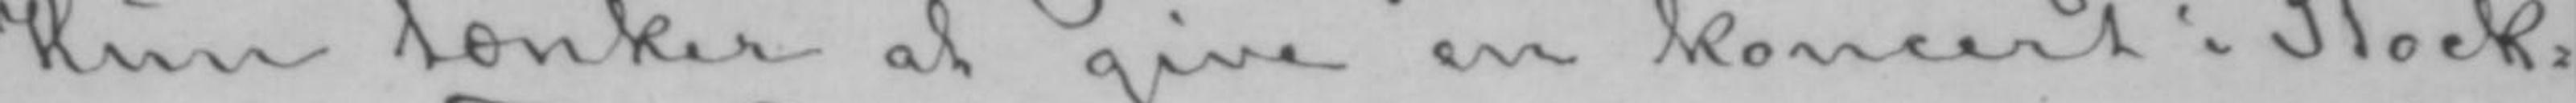Hun tænker at give en koncert i Stock-
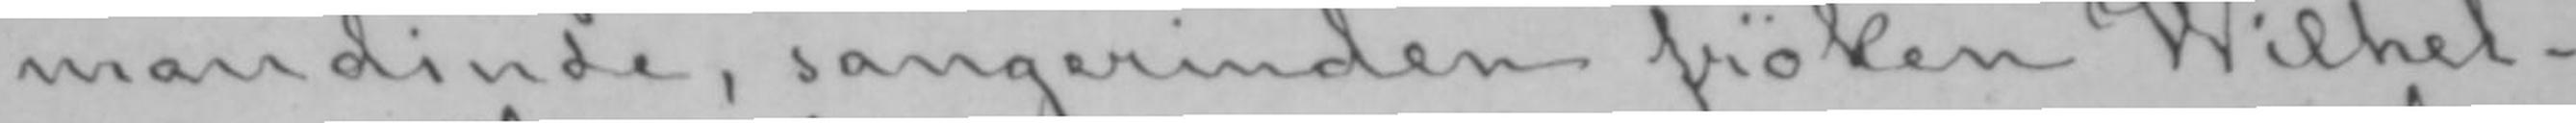mandinde, sangerinden frøken Wilhel-
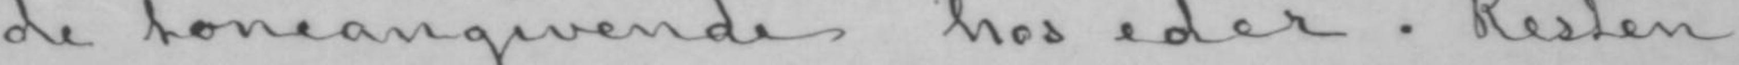de toneangivende hos eder. Resten
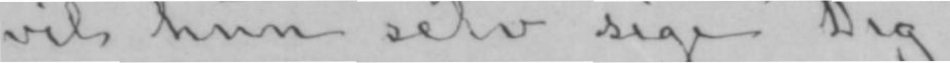vil hun selv sige Dig.
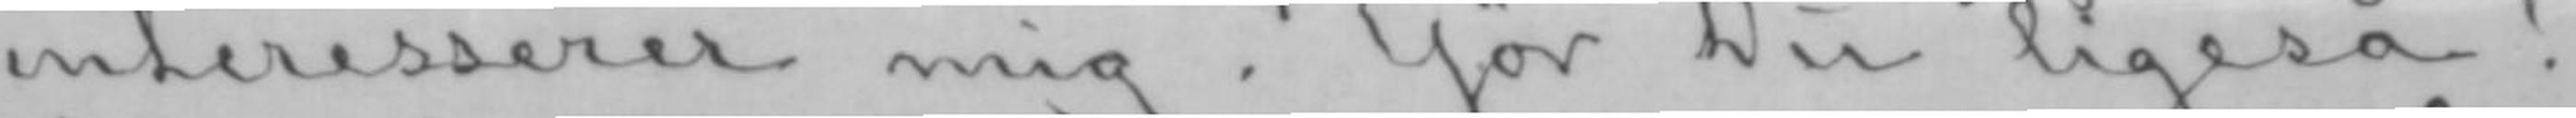interesserer mig. Gør Du ligeså!
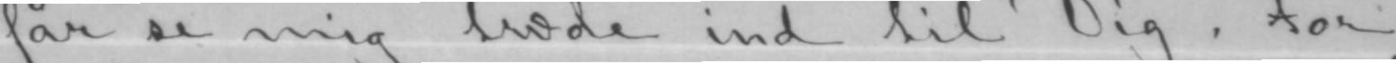får se mig træde ind til Dig. For
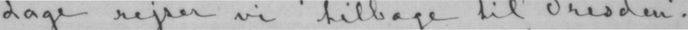dage rejser vi tilbage til Dresden.
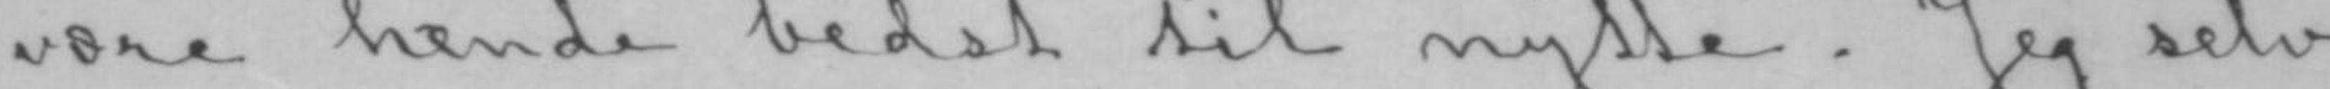være hende bedst til nytte. Jeg selv
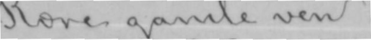Kære gamle ven!
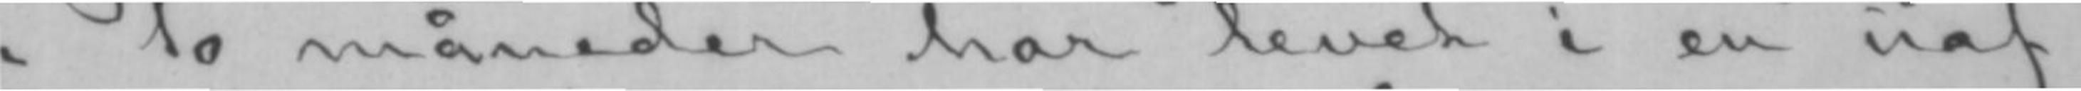i to måneder har levet i en uaf-
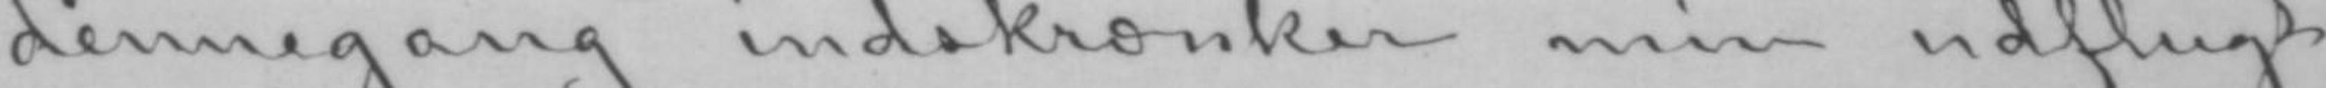dennegang indskrænker min udflugt
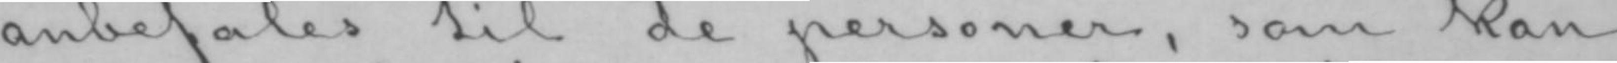anbefales til de personer, som kan
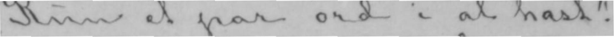Kun et par ord i al hast!
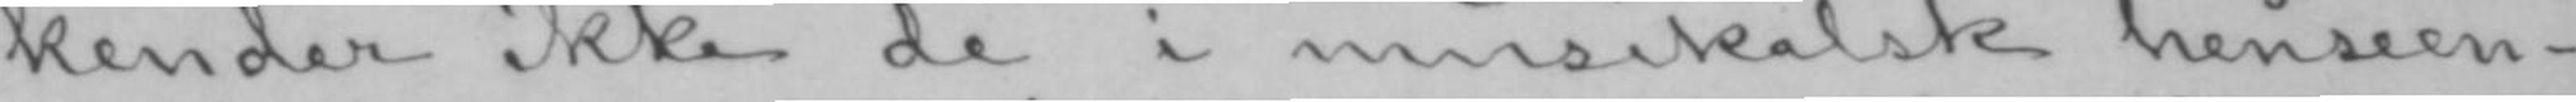kender ikke de i musikalsk henseen-
